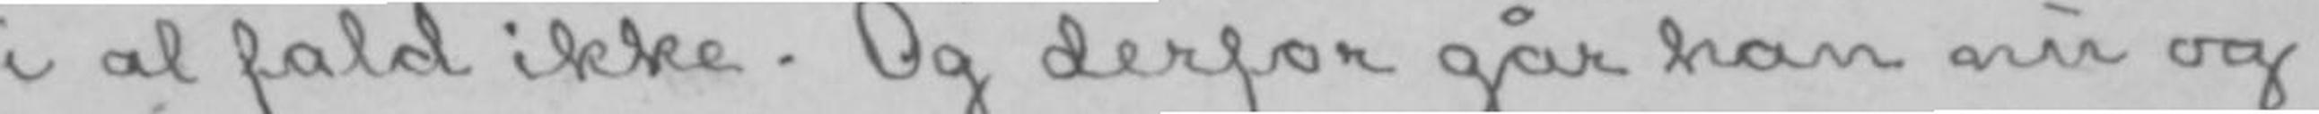i al fald ikke. Og derfor går han nu og
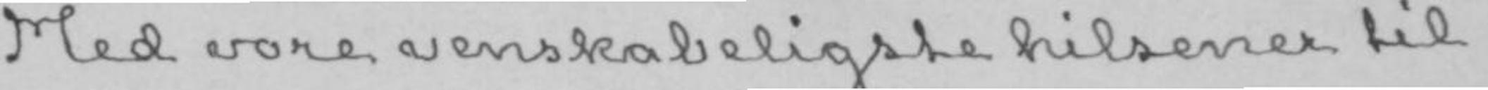Med vore venskabeligste hilsener til
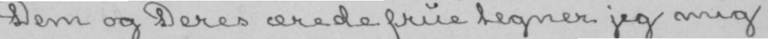Dem og Deres ærede frue tegner jeg mig
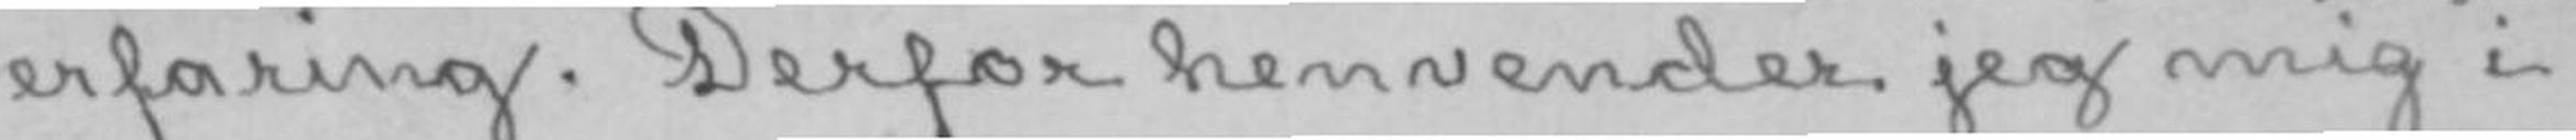erfaring. Derfor henvender jeg mig i
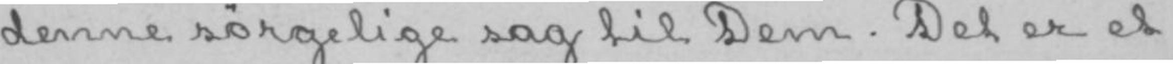denne sørgelige sag til Dem. Det er et
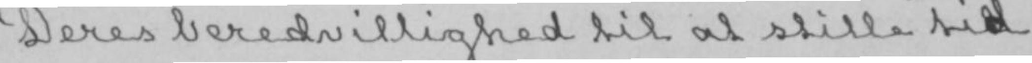Deres beredvillighed til at stille tild
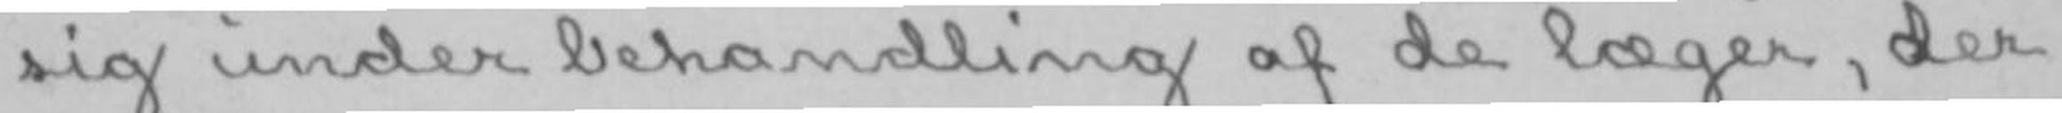sig under behandling af de læger, der
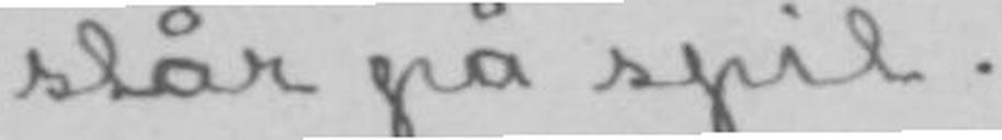står på spil.
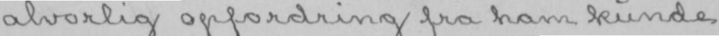alvorlig opfordring fra ham kunde
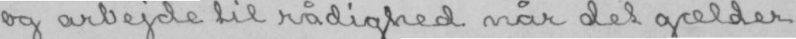og arbejde til rådighed når det gælder
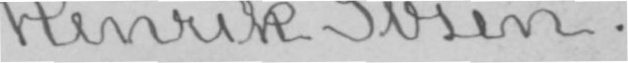Henrik Ibsen.
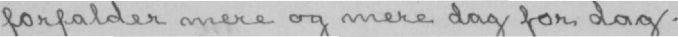forfalder mere og mere dag for dag.
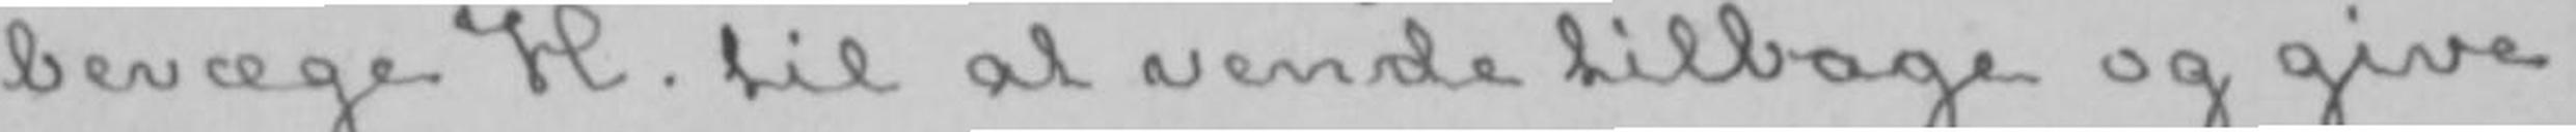bevæge H. til at vende tilbage og give
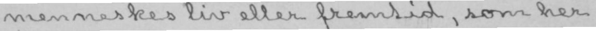menneskes liv eller fremtid, som her
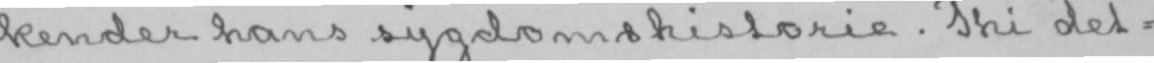kender hans sygdomshistorie. Thi det-
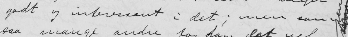godt og interessant i det; men som
